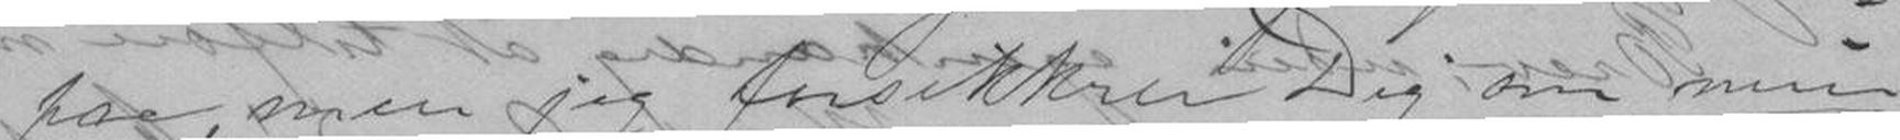paa, men jeg forsikkrer Dig om min
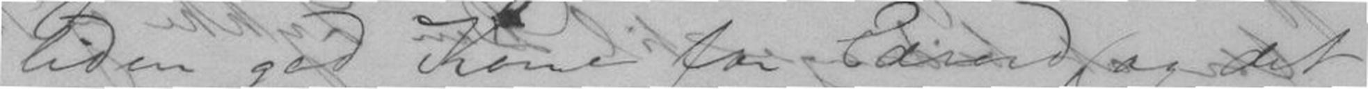liden god Kone for Edvard, og det
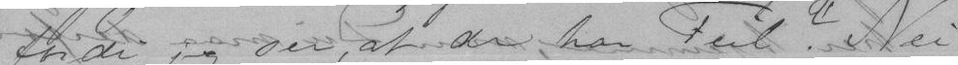fordi jeg ser, at de har Feil? Nei
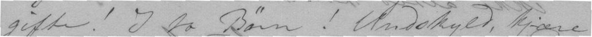gifte! I to Børn! Undskyld, kjære
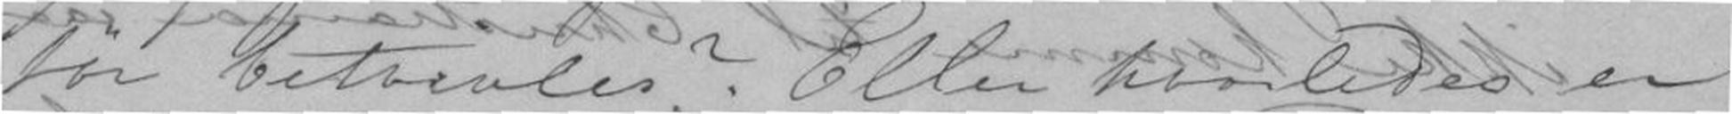tør betvivles? Eller hvorledes er
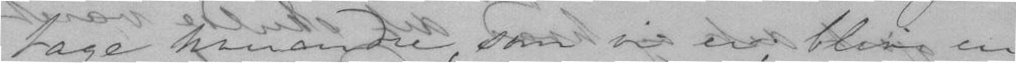tage hverandre, som vi er, blive en
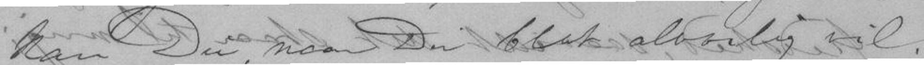kan Du, naar Du blot alvorlig vil;
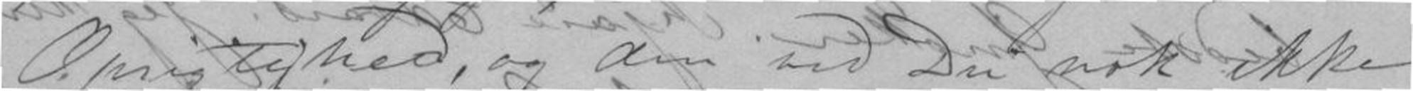Oprigtighed, og den ved Du nok ikke
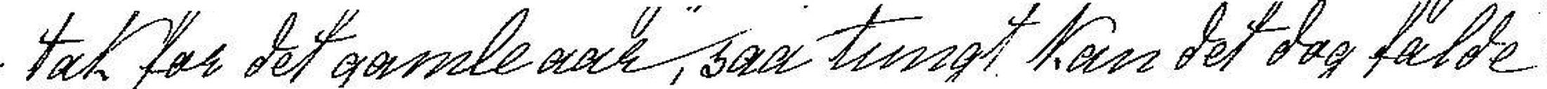tak for det gamle aar, saa tungt kan det dog falde
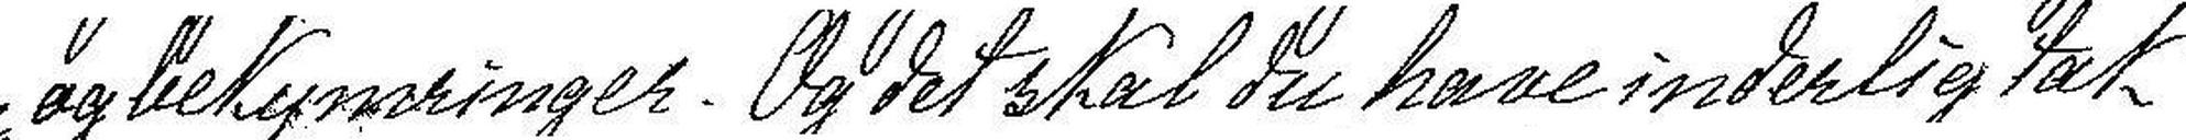og bekymringer. Og det skal du have inderlig tak
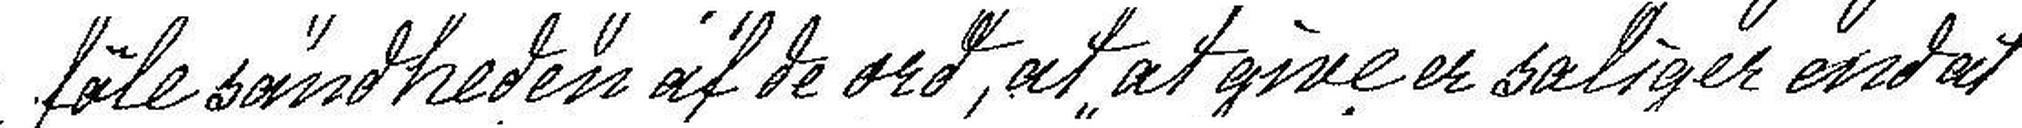føle sandheden af de ord, at at give er saliger end at
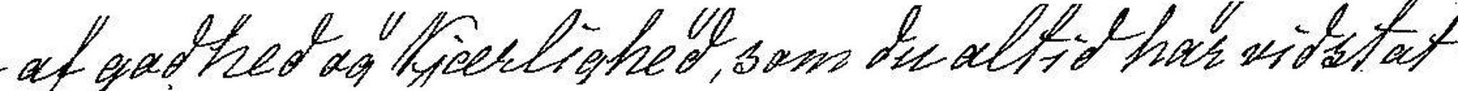af godhed og Kjærlighed, som du altid har vidst at
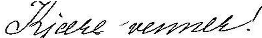Kjære venner!
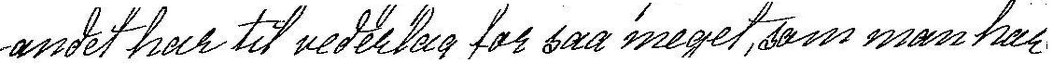andet har til vederlag for saa meget, som man har
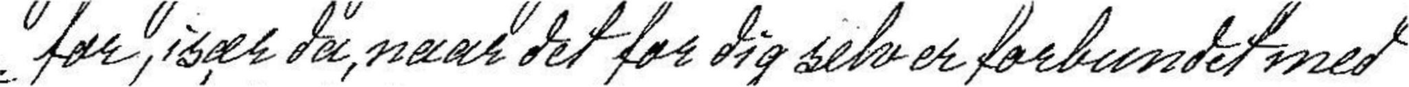for, især da, naar det for sig selv er forbundet med
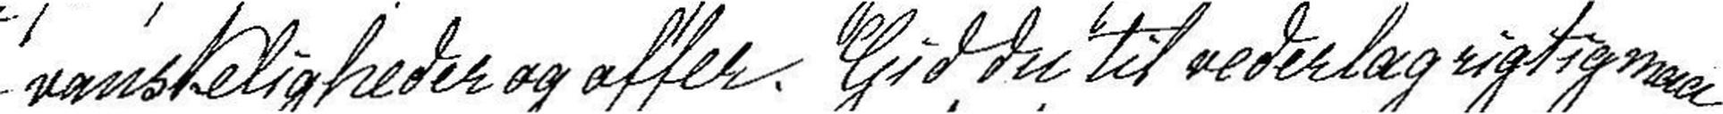vanskeligheder og offer. Gid du til vederlag rigtig maa
